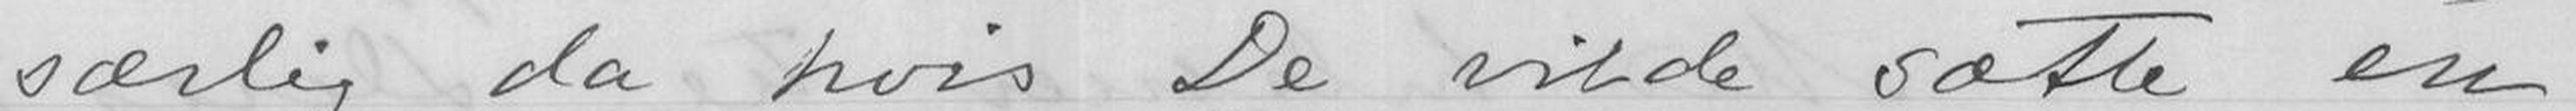særlig da hvis De vilde sætte en
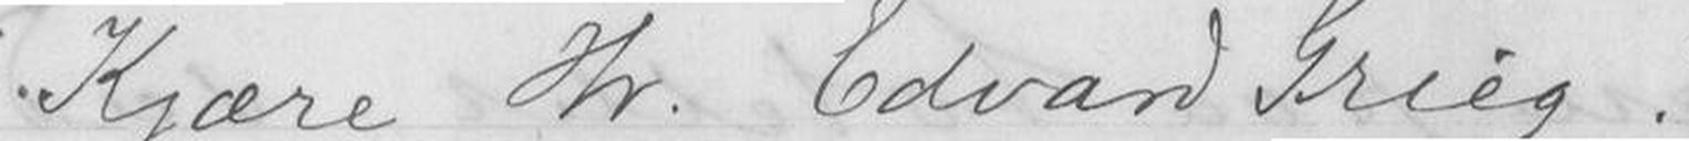Kjære Hr. Edvard Grieg!
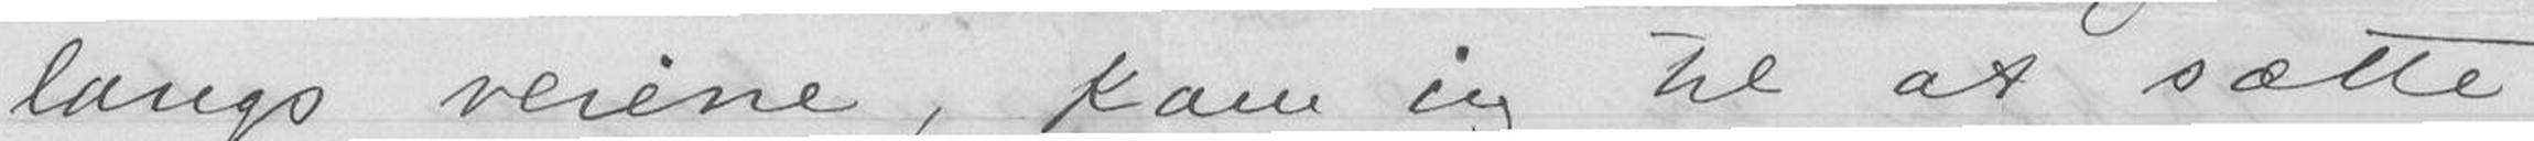langs veiene, kom jeg til at sætte
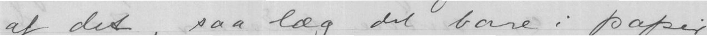af det, saa læg det bare i papir
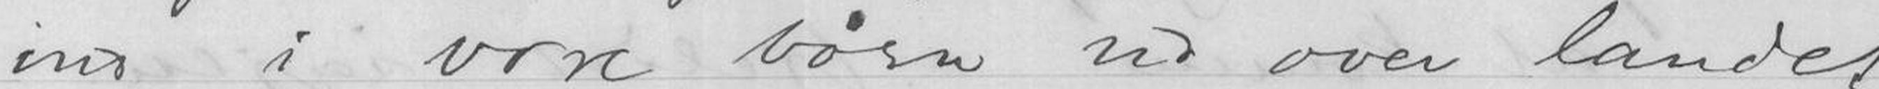ind i vore børn ud over landet,
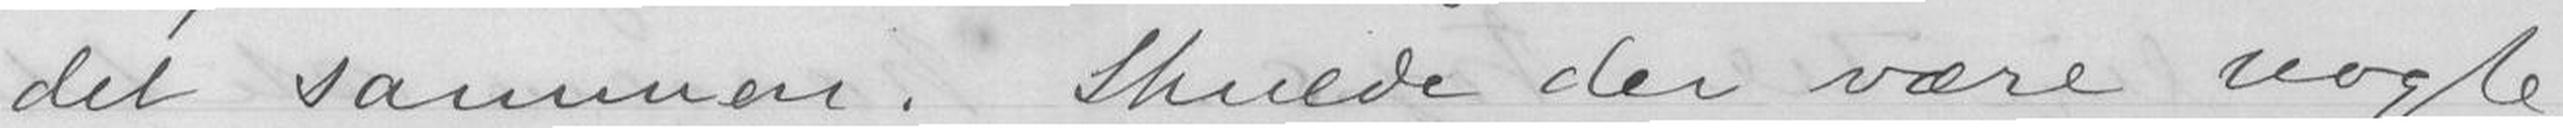det sammen. Skulde der være nogle
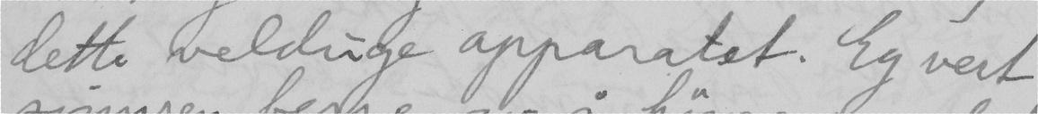dette velduge apparatet. Eg vert
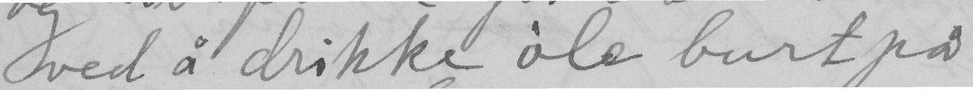med å drikke öle burt på
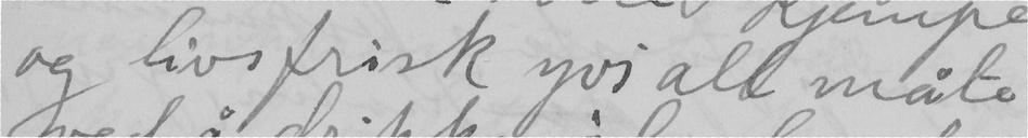og livsfrisk yvi all måte
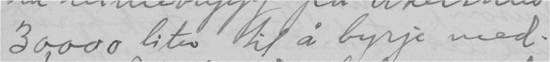30,000 liter til å byrje med.
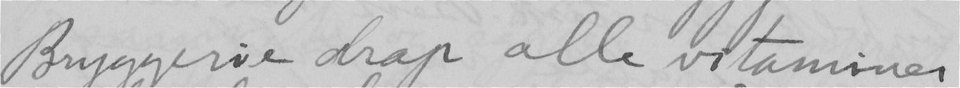Bryggerie drap alle vitaminar
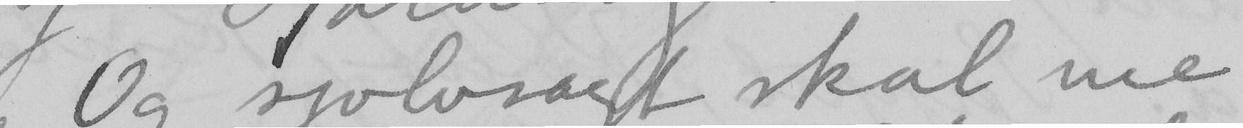Og sjölvsagt skal me
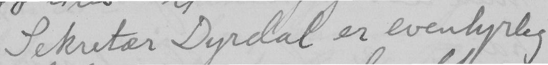Sekretær Dyrdal er eventyrleg
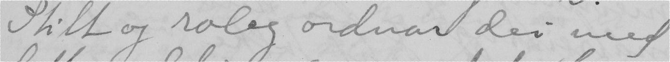Stilt og roleg ordnar dei med
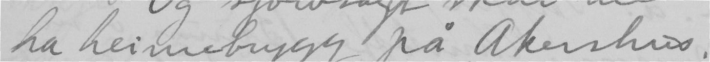ha heimebrygg på Akershus.
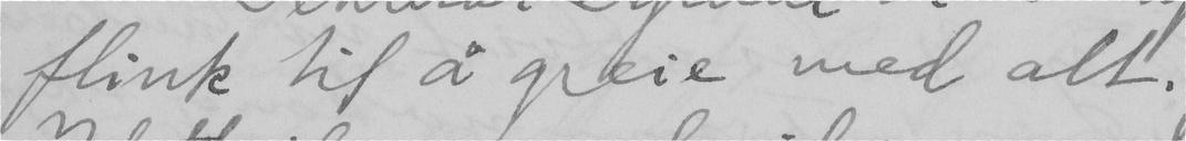flink til å greie med alt.
